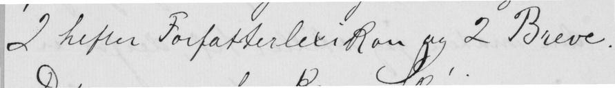2 hefter Forfatterlexikon og 2 Breve.
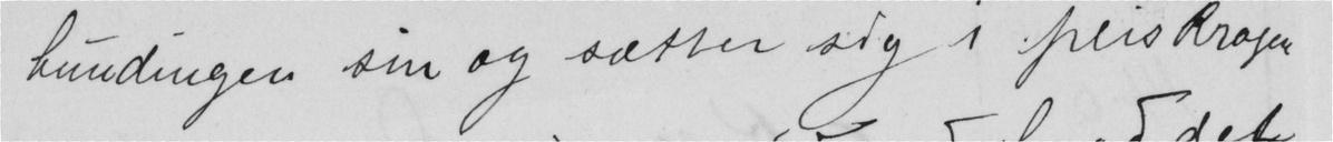hundingen sin og sætter sig i pelskragen
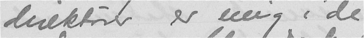direktører er enig i de
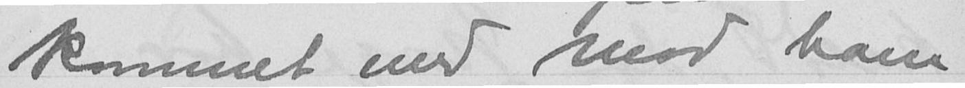kommet med mod ham
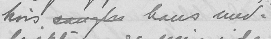hvis saugbr hans med-
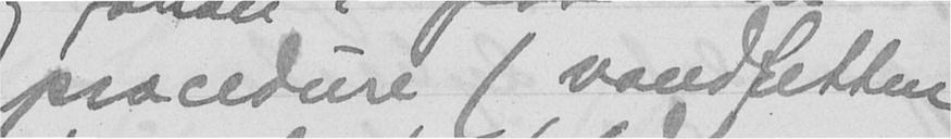procedure (vandtetten
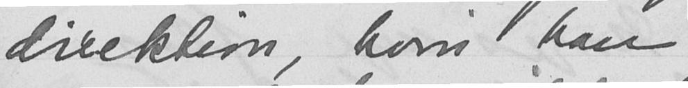direktion, hvor han
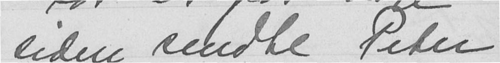siden sendte Peter
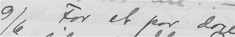9/6 For et par dage
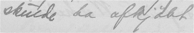skulde ha afkjøbt
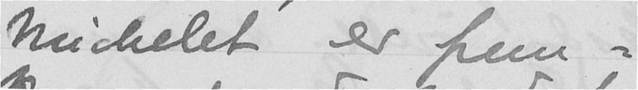Michelet er frem-
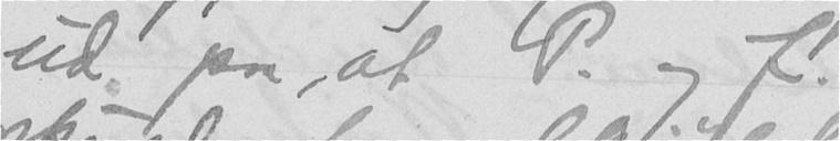ud paa, at P. og J.
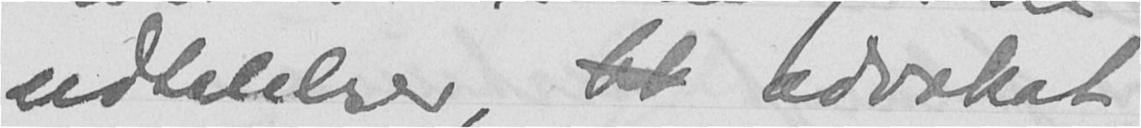udtalelser, bl advokat
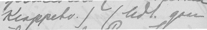Krappeto.) (Udt. gaar
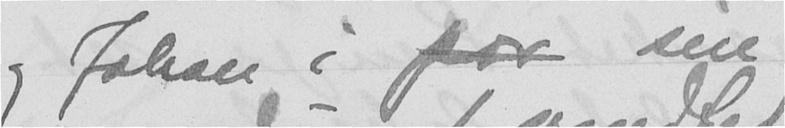og Johan i paa sin
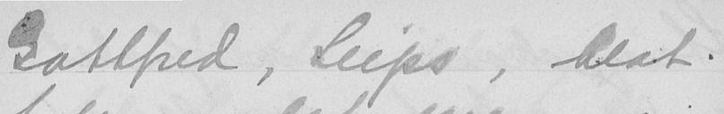Gottfred, Seips, blot
